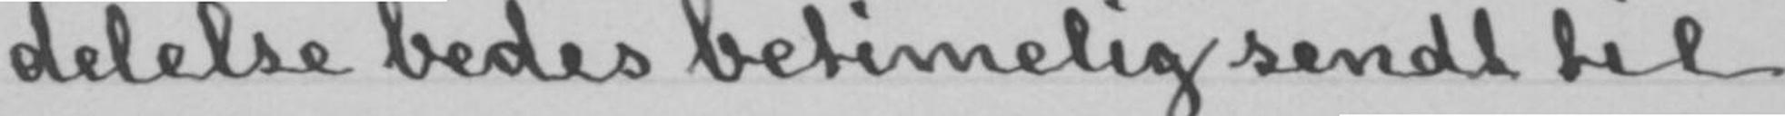delelse bedes betimelig sendt til
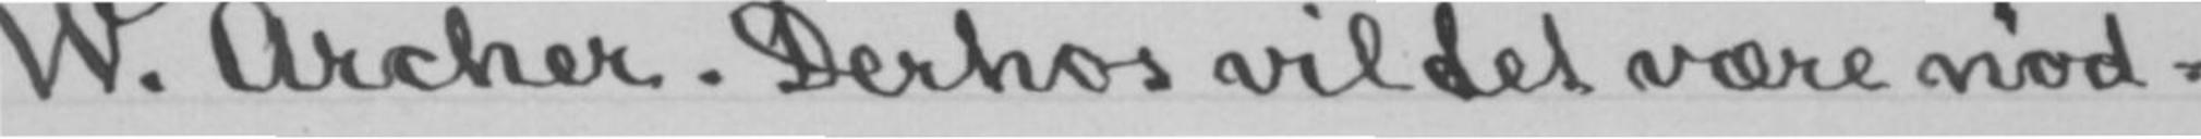W. Archer. Derhos vil det være nød-
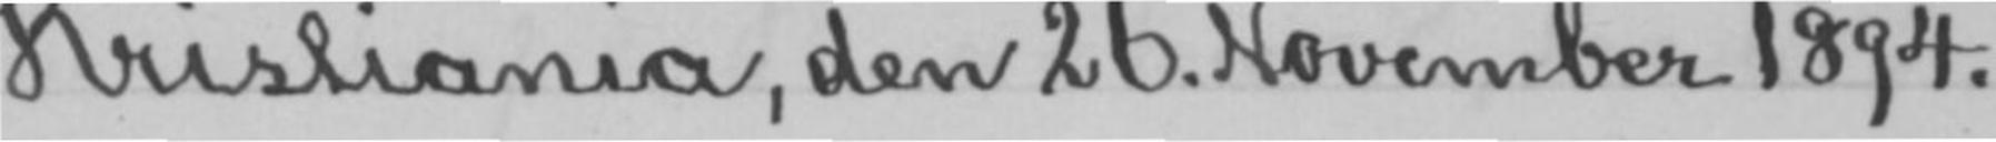Kristiania, den 26. November 1894.
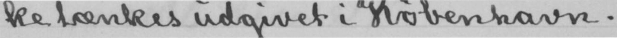ke tænkes udgivet i København.
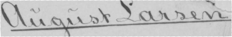August Larsen
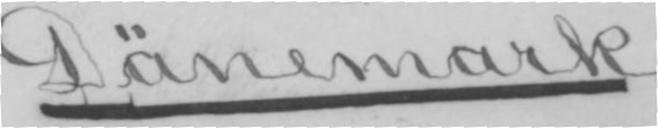Dänemark.
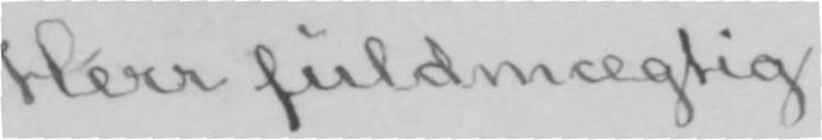Herr fuldmægtig
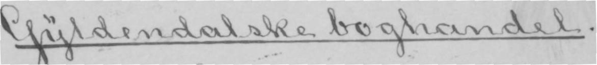Gyldendalske boghandel.
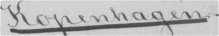Kopenhagen.
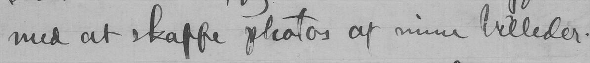med at skaffe photos af mine billeder.
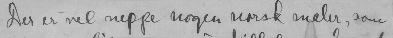Der er vel neppe nogen norsk maler, som
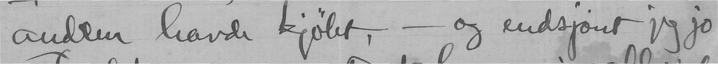anden havde kjøbt, - og endsjønt jeg jo
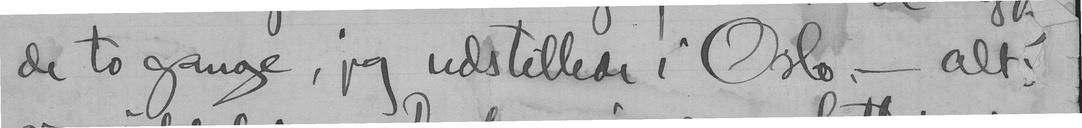de to gange, jeg udstillede i Oslo, - alt
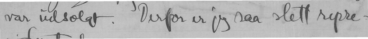var udsolgt. Derfor er jeg saa slett repre-
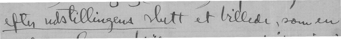efter udstillingens slutt et billede, som en
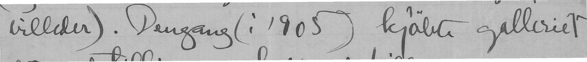billeder). Dengang (i 1905) kjøbte galleriet
